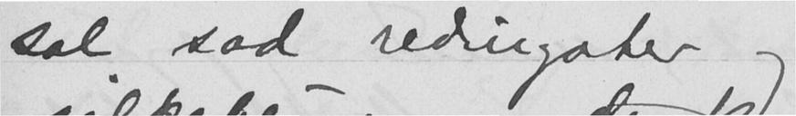sal sad redingoter og
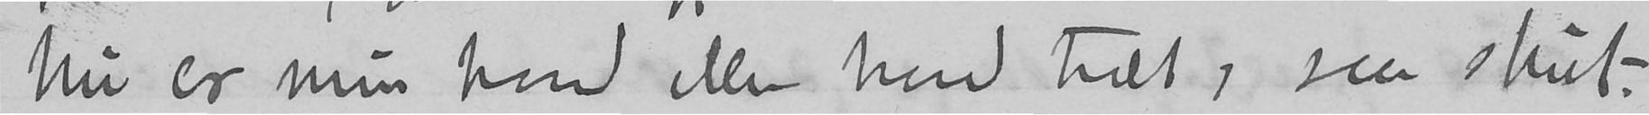Nu er min hand eller hode træt, saa slut-
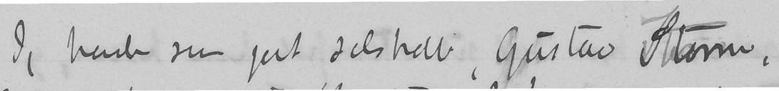Jeg havde saa godt selskab, Gustav Storm,
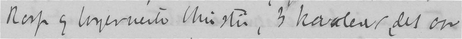Rorp og borgermester Christir, 3 kavalerer, det var
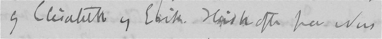og Elisabeth og Erik. Husk ofte paa eders
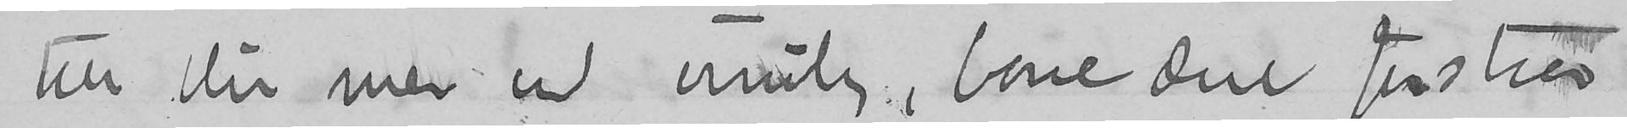ten blir mer end umulig, bare dere forstaar
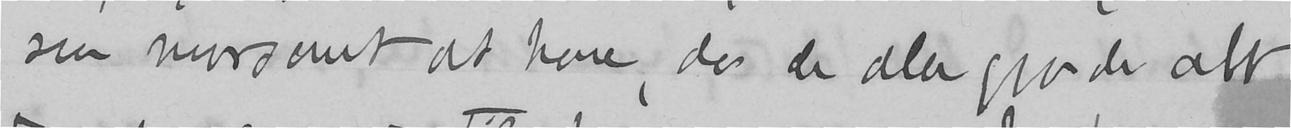saa morsomt at have, da de alle gjorde alt
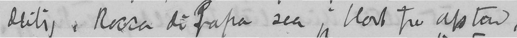deilig, Rocca di Papa saa jeg blot fra afstand,
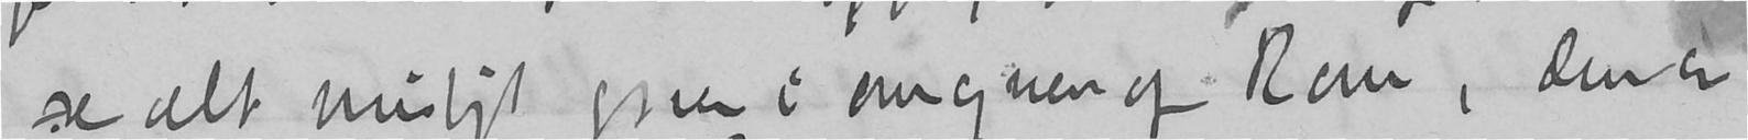se alt muligt ogsaa i omegnen af Rom, den er
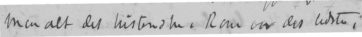Men alt det tristenske. Rom var det bedste,
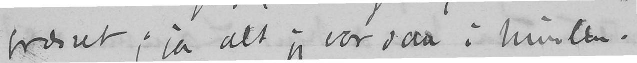græsset; ja alt jeg var saa i himlen.
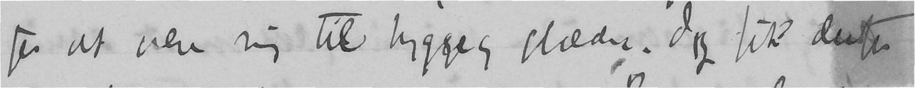for at være mig til hygge og glæde. Jeg fik derefter
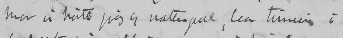hvor vi hørte gjøg og nattergal, laa timevis i
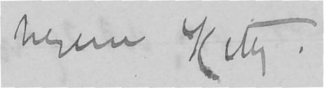hengivne Kitty.
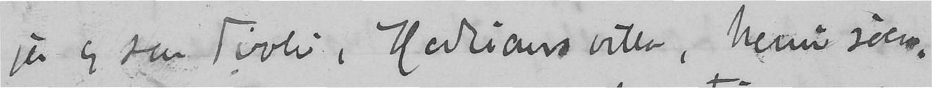ja og saa Tivoli, Hadrians villa, Nemisøen,
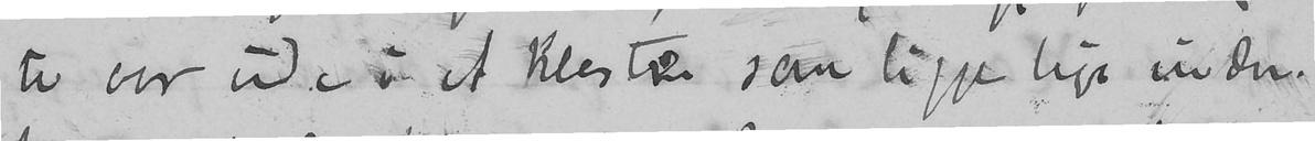ta var ude i et kloster som ligge lige under.
